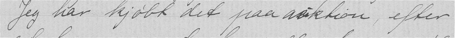Jeg har kjøbt det paa auktion, efter
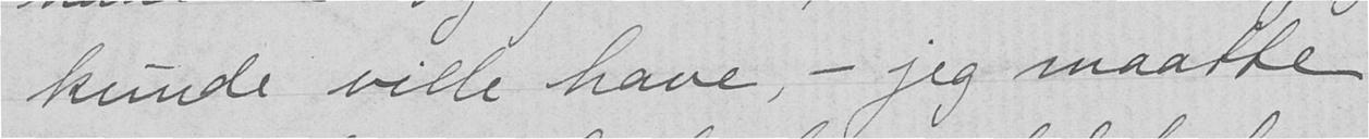kunde ville have, - jeg maatte
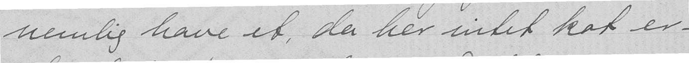nemlig have et, da her intet kot er -
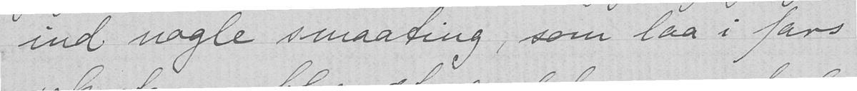ind nogle smaating, som laa i fars
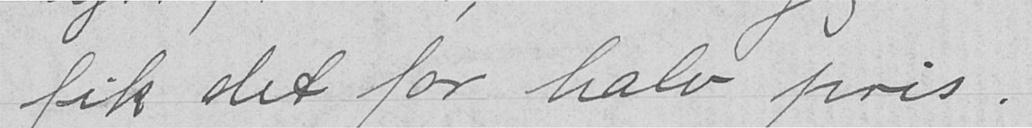fik det for halv pris.
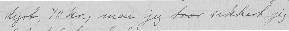dyrt, 70 kr.; men jeg tror sikkert, jeg
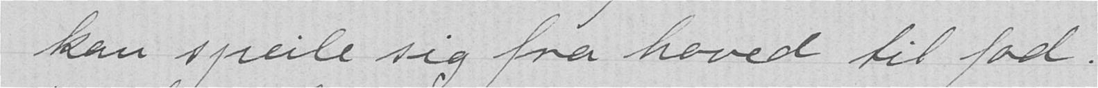kan speile sig fra hoved til fod.
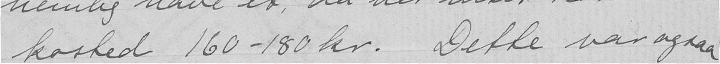kosted 160-180 kr. Dette var ogsaa
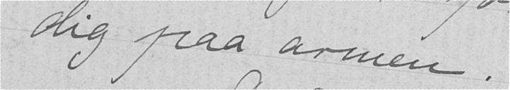dig paa armen.
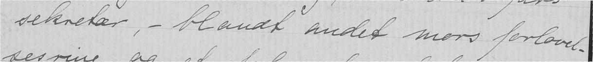sekretær, - blandt andet mors forlovel-
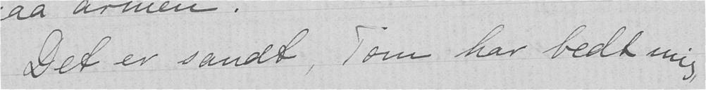Det er sandt, Tom har bedt mig,
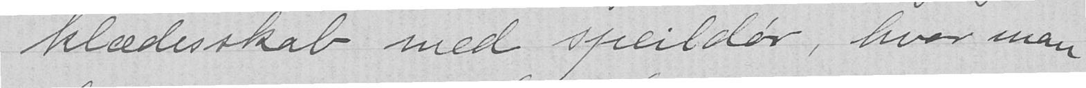klædesskab med speildør, hvor man
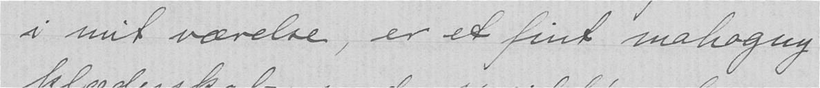i mit værelse, er et fint mahogny
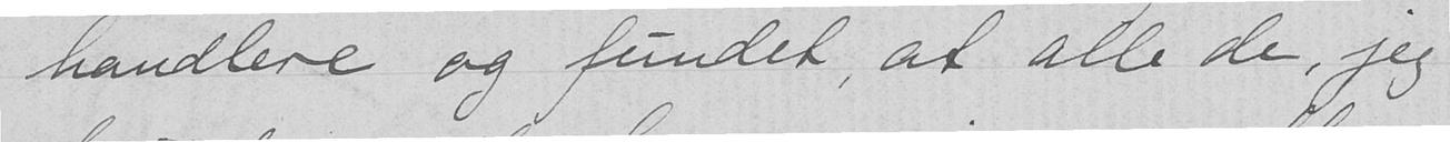handlere og fundet, at alle de, jeg
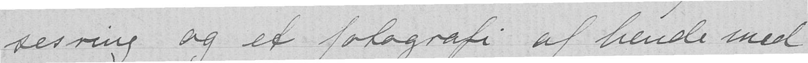sesring og et fotografi af hende med
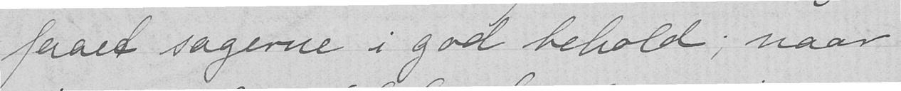faaet sagerne i god behold; naar
